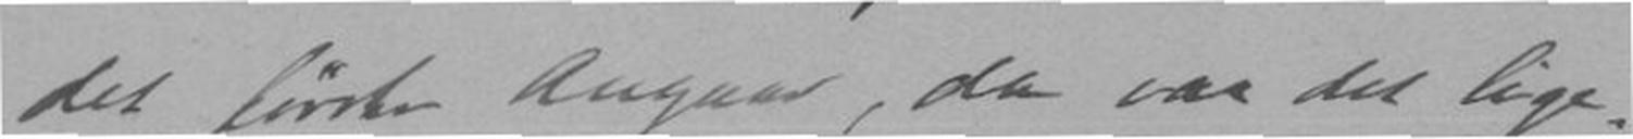det først Angaar, da var det lige-
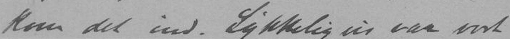kom det ind. Lykkeligvis var vort
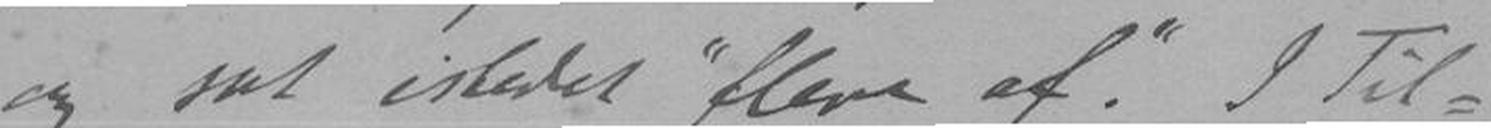og sat istedet "flere af". I Til-
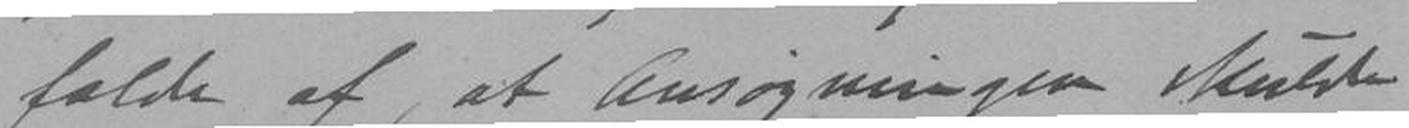fælde af, at Ansøgningen skulde
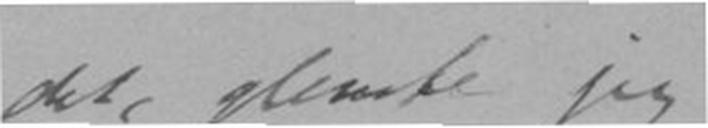det, glemte jeg
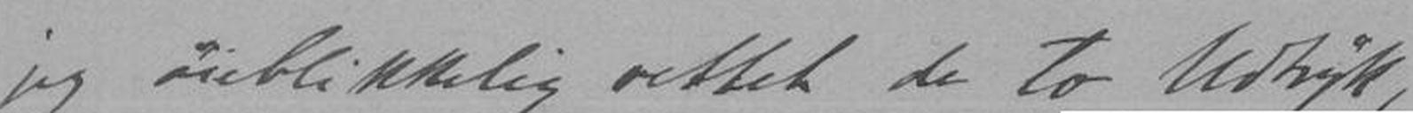jeg øieblikkelig rettet de to Udtryk,
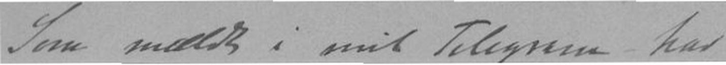Som mældt i mit Telegram har
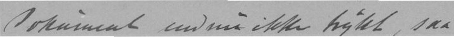Dokument endnu ikke trykt, saa
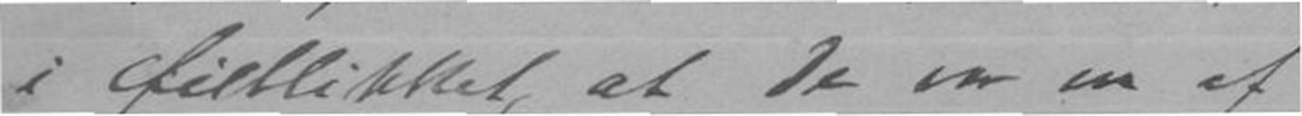i Øieblikket, at De var en af
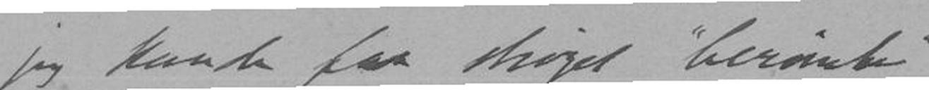jeg kunde faa strøget "berønte"
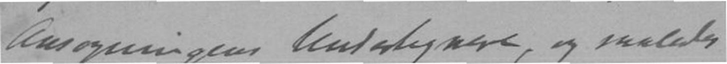Ansøgningens Undertegnere, og saaledes
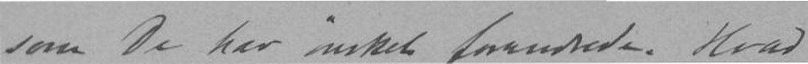som De har ønsket forandrede. Hvad
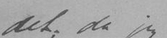det; da jeg
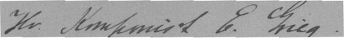Hr Komponist E. Grieg!
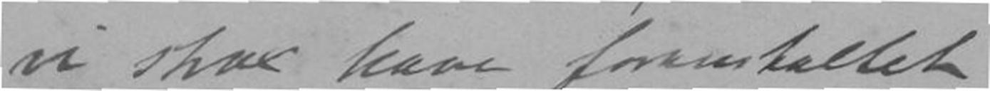vi strax have foranstallet
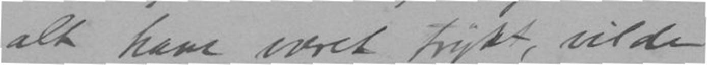alt have været trykt, vilde
